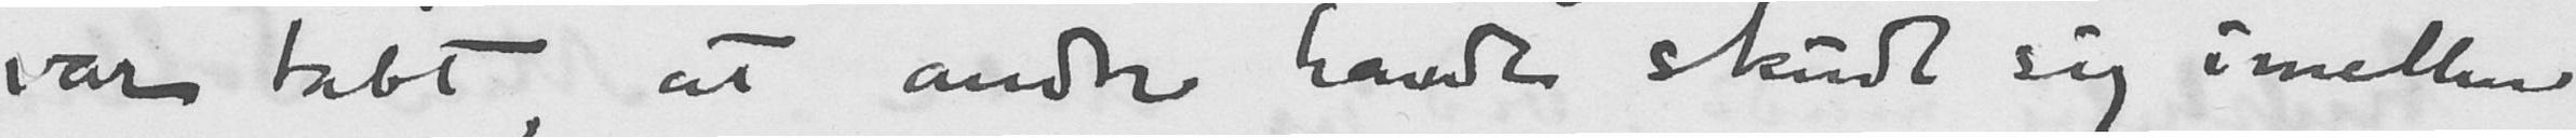var tabt, at andre havde skudt sig imellem
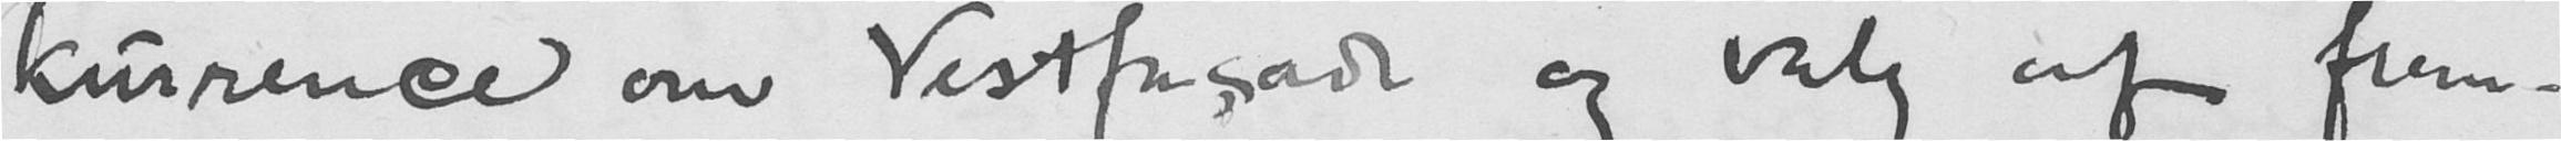kurence om Vestfacade og valg af frem-
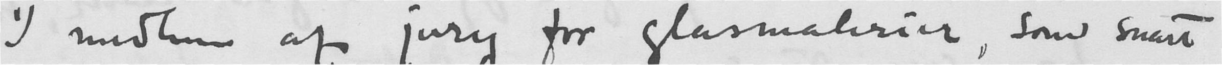1) medlem af jury for glasmalerier, som snart
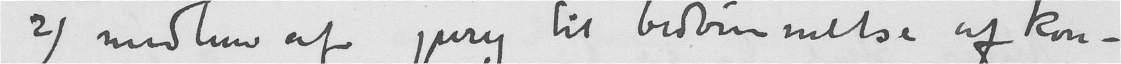2) medlem af jury til bedømmelse af kon-
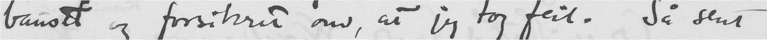bauset og forsikret om, at jeg tog feil. Så sent
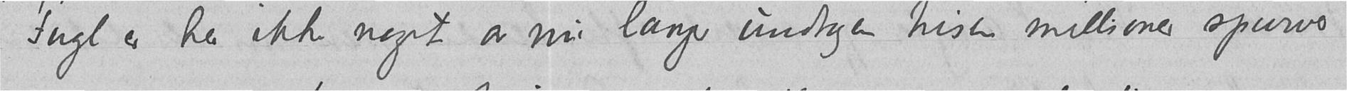Fugl er her ikke noget av nu længet undtagen tusen millioner spurver
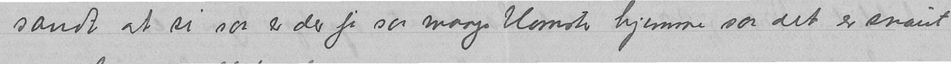sandt at si saa er der jo saa mange blomster hjemme saa det er snaut
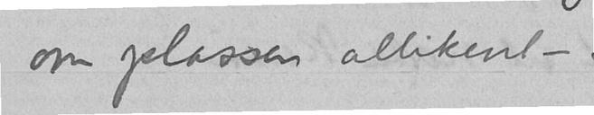om plassen allikevel -.
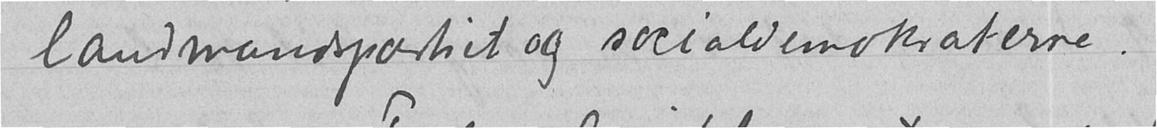landmandspartiet og socialdemokraterne.
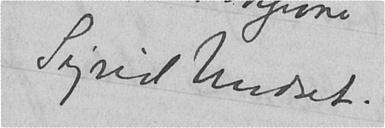Sigrid Undset.
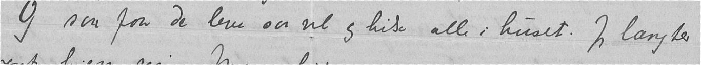Og saa faar De leve saa vel og hilse alle i huset. Jeg længter
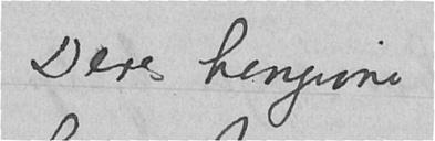Deres hengivne
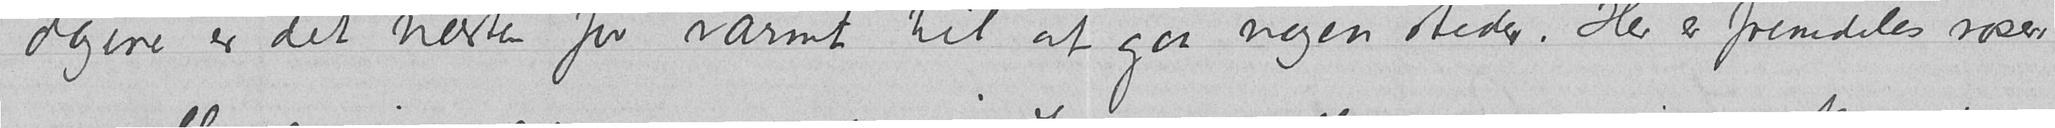dagene er det næsten for varmt til at gaa nogen steder. Her er fremdeles roser
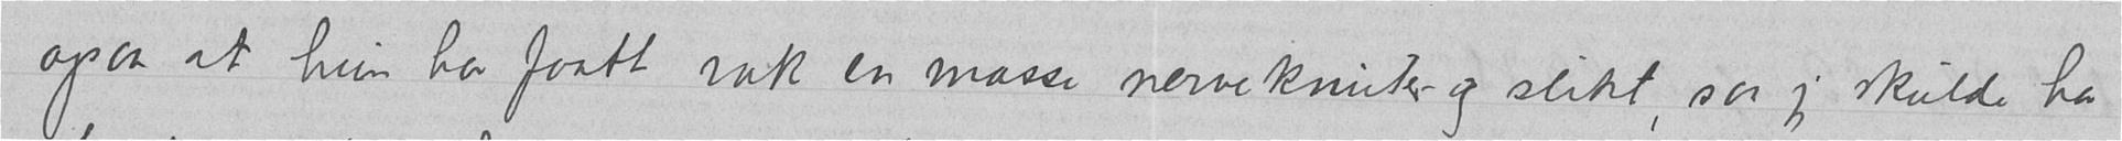ogsaa at hun har faatt væk en masse nerveknuter og slikt, saa jeg skulde ha
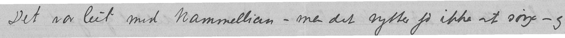Det var leit med kammelliaen – men det nytter jo ikke at sørge – og
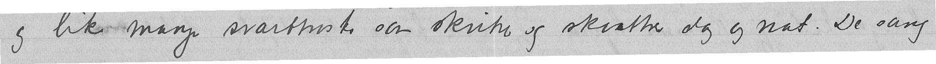og like mange svarttroster som skriker og skvattrer dag og nat. De sang
